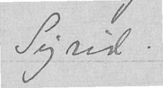Sigrid.
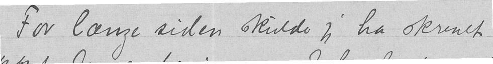For længe siden skulde jeg ha skrevet
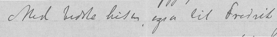Med bedste hilsen, ogsa til Fredrik
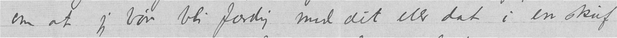om at jeg bør bli færdig med dit eler dat i en skuf
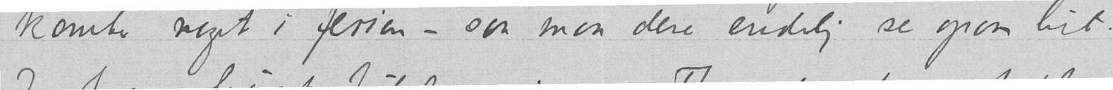kanter noget i ferien – saa maa dere endelig se opom hit.
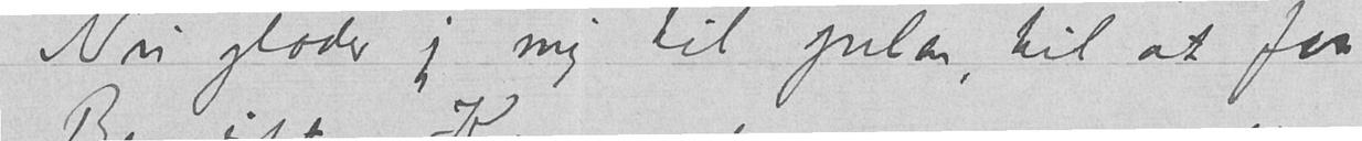Nu glæder jeg mig til julen, til at faa
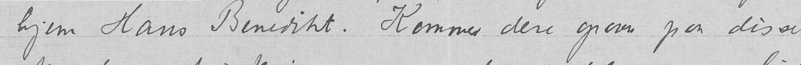hjem Hans Benedikt. Kommer dere opover paa disse
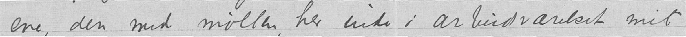ene, den med møllen, her inde i arbeidsværelset mit
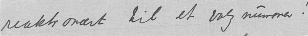reaktionært til et valgnummer!
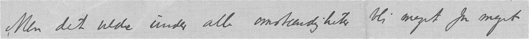Men det vilde under alle omstændigheter bli meget for meget
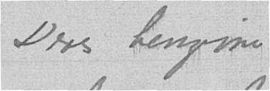Deres hengivne
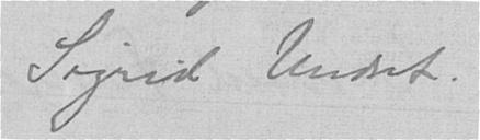Sigrid Undset.
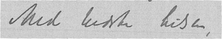Med bedste hilsen,
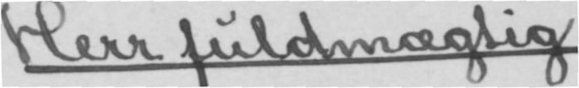Herr fuldmægtig
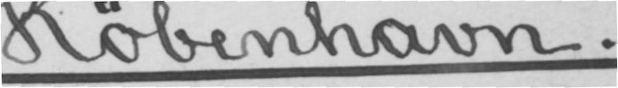Københaven.
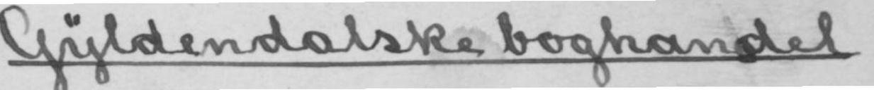Gyldendalske boghandel,
